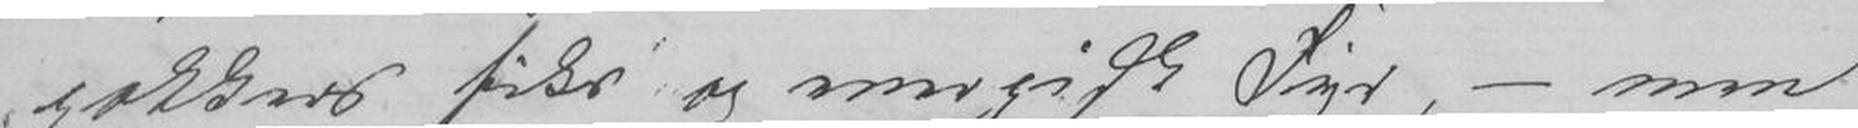pokkers fiks og energisk Fyr, - men
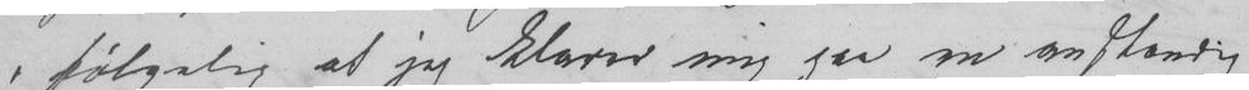følgelig at jeg klarer mig paa en anstendig
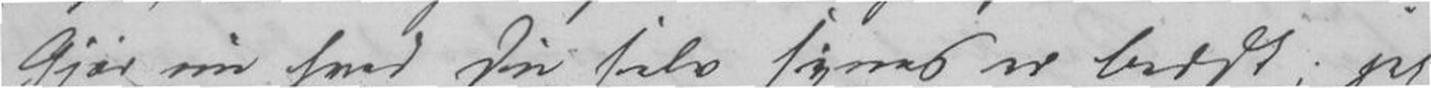Gjør nu hvad Du selv synes er bedst; jeg
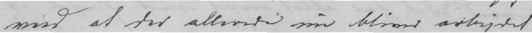ved at der allerede nu bliver arbejdet
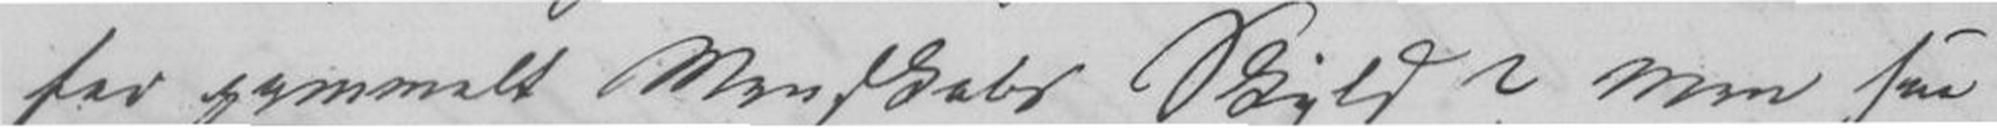for gammelt Venskabs Skyld? Men saa
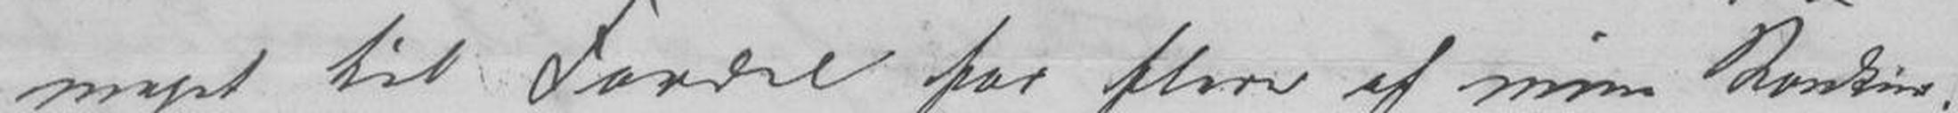meget til Fordel for flere af mine Konkur-
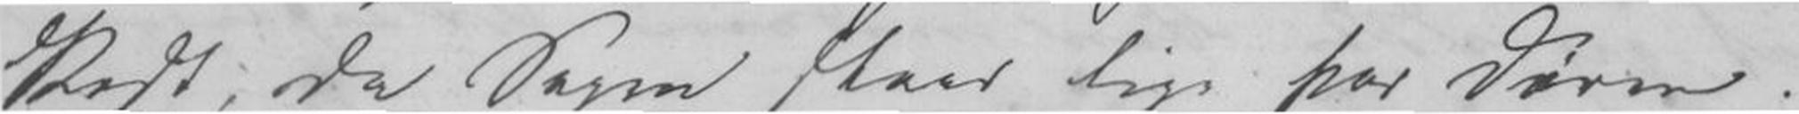post, da Dagen staar lige for Døren.
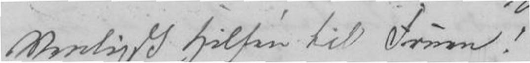Vennligst hilsen til Fruen!
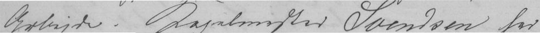Arbeide. Kapelmester Svendsen har
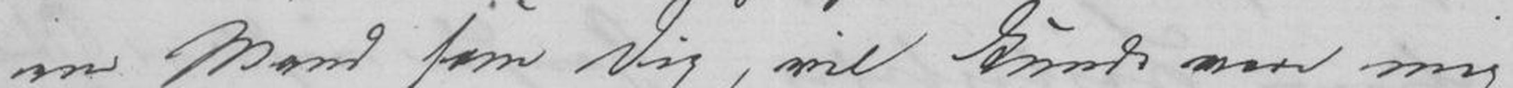en Mand som Dig, vil kunde være mig
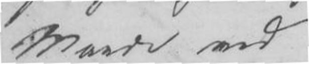Maade med
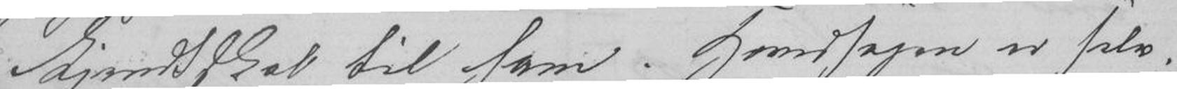kjendtskab til ham. Hovedsagen er selv-
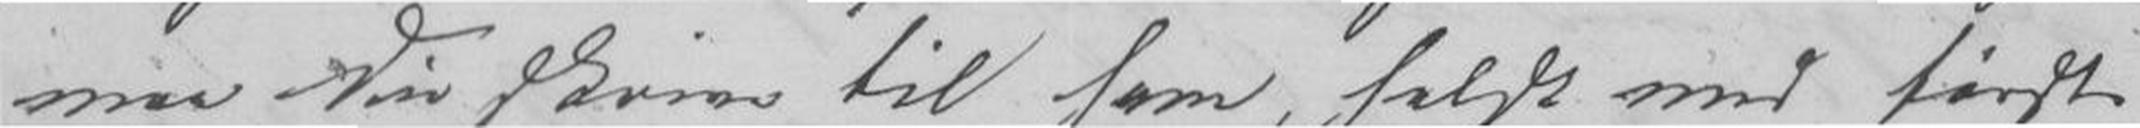maa Du skrive til ham, helst med første
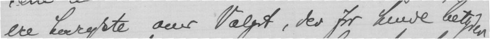ere over Valget, der for kunde betyder
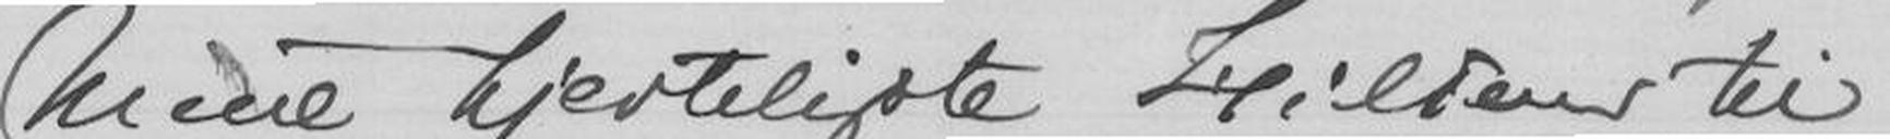Mine hjerteligste hilsener til
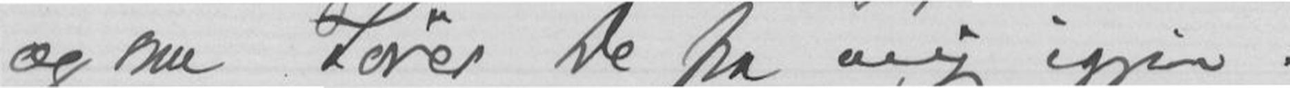og nu hører De fra mig igjen.
